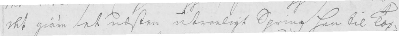det giøre et næsten utroeligt Spring hen til Top-
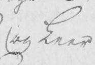og Leer
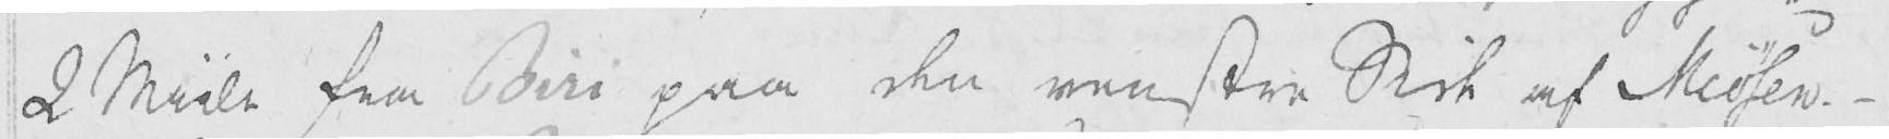2 Miile fra Biri paa den venstre Side af Miøsen. -
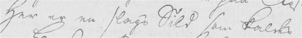her er en slags Sild som kaldes
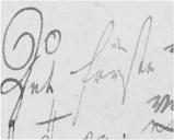Det første
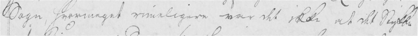Sogn, hvormeget rimeligere var det ikke at det Stykke
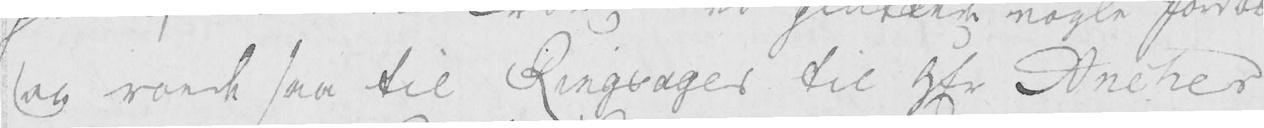og roede saa til Ringsager til Hr Ancher
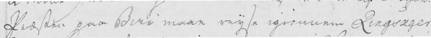Præsten paa Biri maae reyse igiennem Ringsager
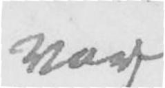var
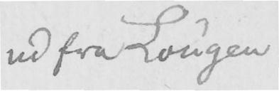ud fra Lougen
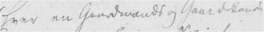hver en Gaardmands og Gaardkones
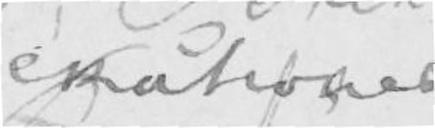ekationer
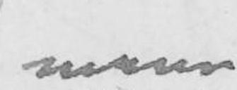mine
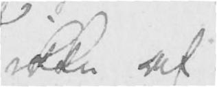ikke af
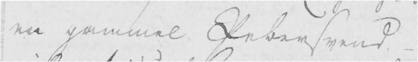en gammel Pebersvend. -
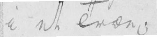i et Træe;
